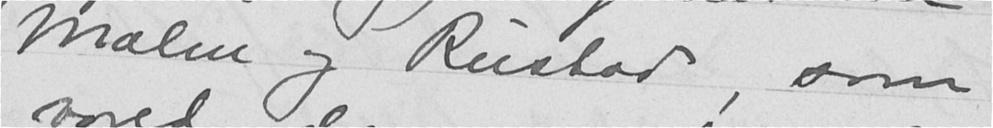Malm og Rustad, som
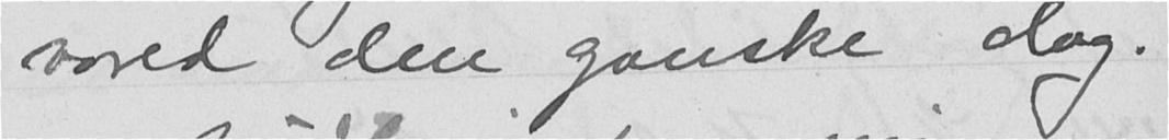vared den ganske dag.
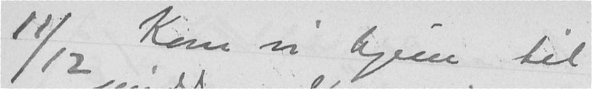11/12 Kom vi hjem til
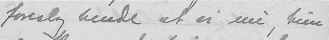foreslog hende at vi nu, hun
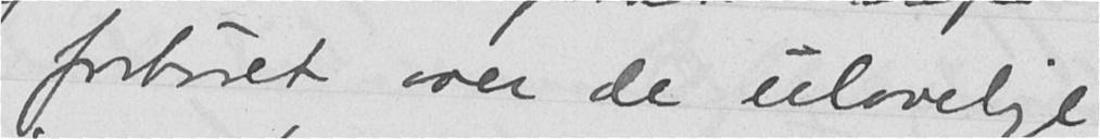forhøret over de ulovelige
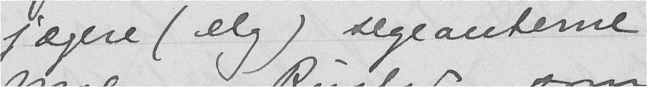jægere (elg) segeanterne
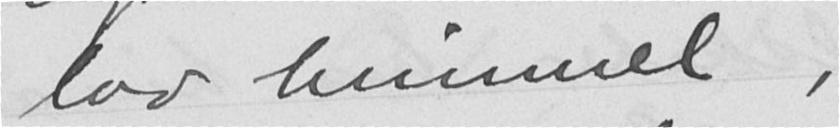lod himmel,
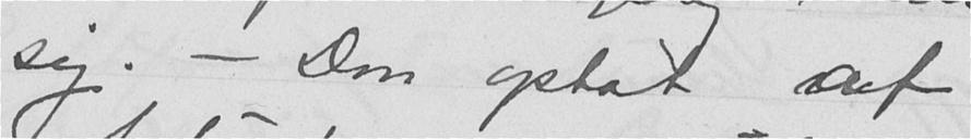sig. - Don optat af
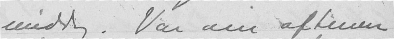middag. Var om aftenen
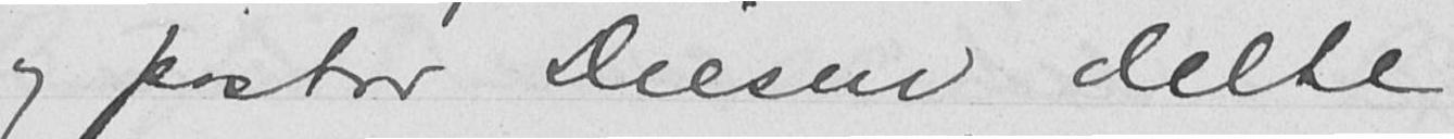og pastor Diesen delte
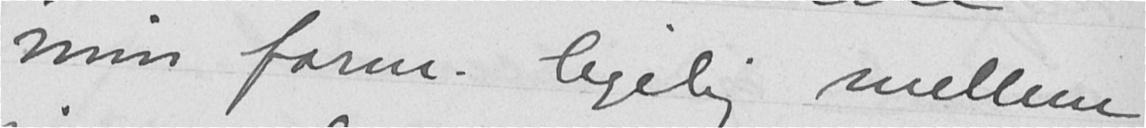min form. ligelig mellem
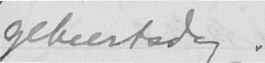geburtsdag.
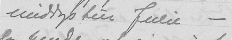middagstur Julie -
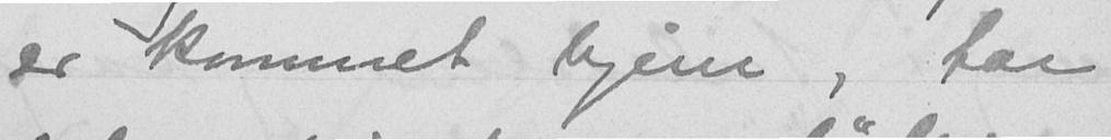er kommet hjem, tar
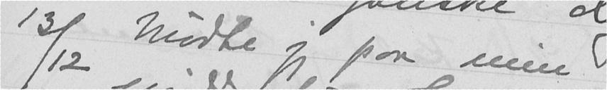13/12 Mødte jeg paa min
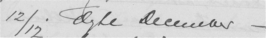12/12 Ægte December -
